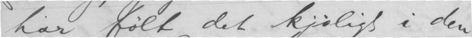har følt det kjøligt i den
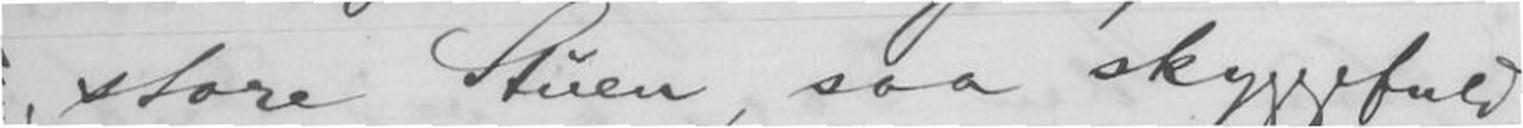store Stuen, saa skyggefuld
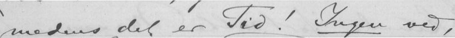medens det er Tid! Ingen ved,
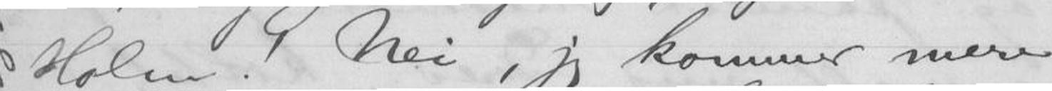Holm! Nei, jeg kommer mere
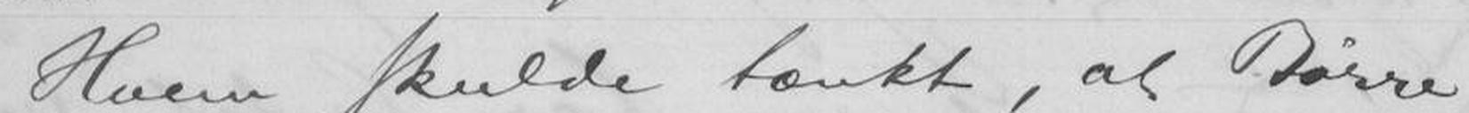Hvem skulde tænkt, at Børre
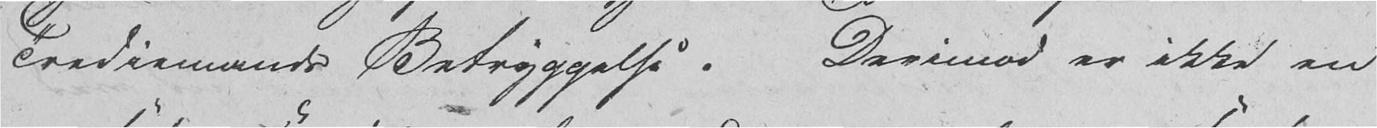Trediemands Betryggelse. Derimod er ikke en
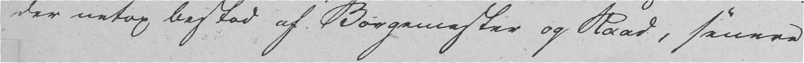der netop bestod af Borgemester og Raad, senere
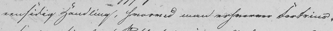eensidig Handling, hvorved man erhverver Fortrins-
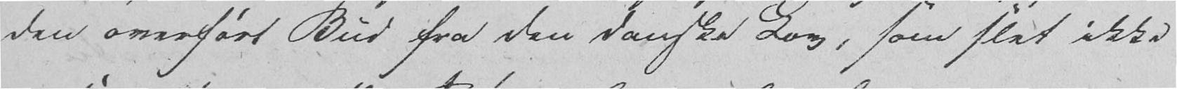den overført Bud fra den danske Lov, som slet ikke
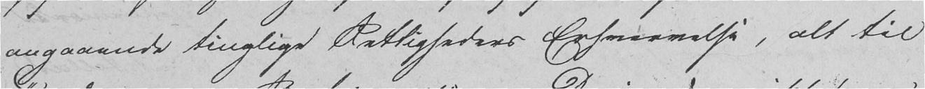angaaende tinglige Rettigheders Erhvervelse, alt til
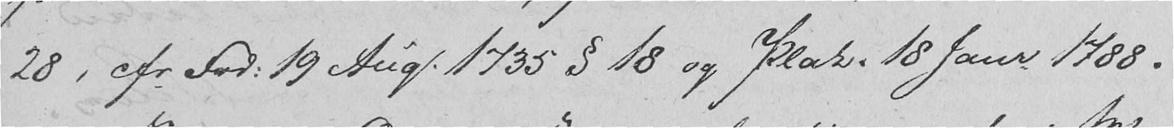28, cfr Frd. 19 Aug. 1735 § 18 og Plak. 18 Janr 1788.
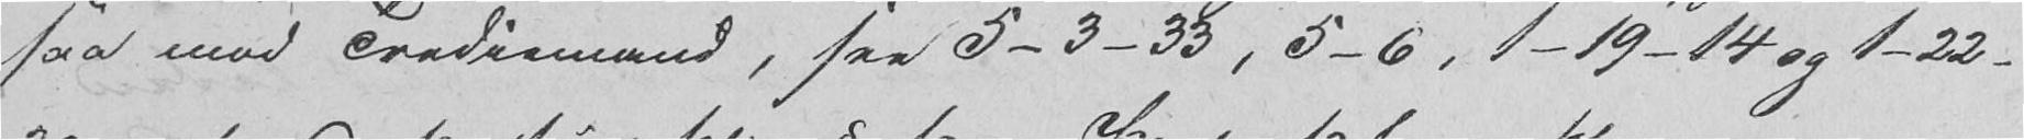saa mod Trediemand, see 5-3-33, 5-6, 1-19-14 og 1-22-
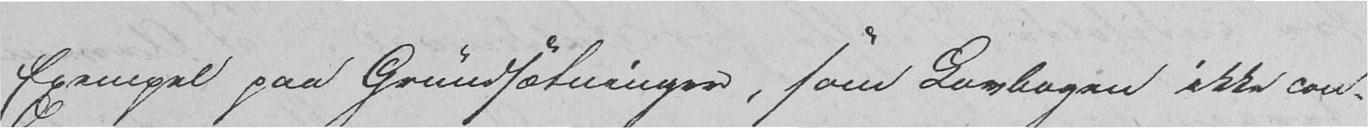Exempel paa Grundsætninger, som Lovbogen ikke con-
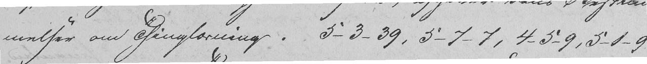melser om Thinglæsning. 5-3-39, 5-7 7, 4-5-9, 5-1-9
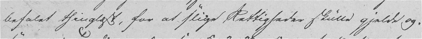befalet thinglæst, for at slige Rettigheder skulle gjelde og-
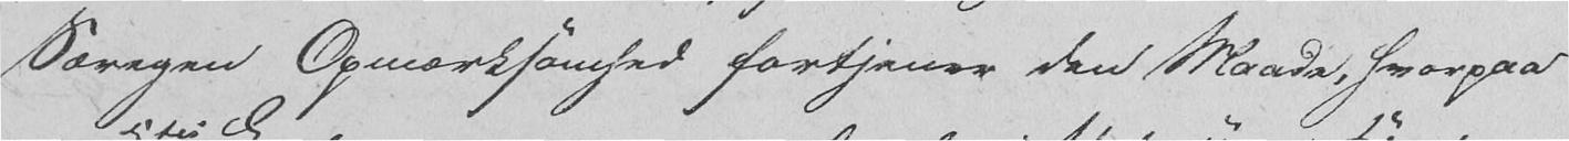Særegen Opmærksomhed fortjener den Maade, hvorpaa
